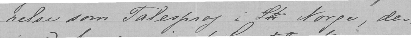relse som Talesprog i Sk Norge, der-
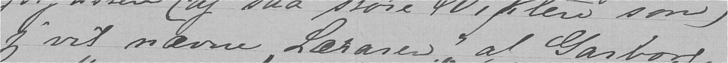jeg vil nævne Læraren af Garborg
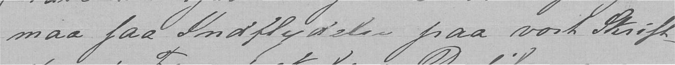maa faa Indflydelse paa vort Skrift-
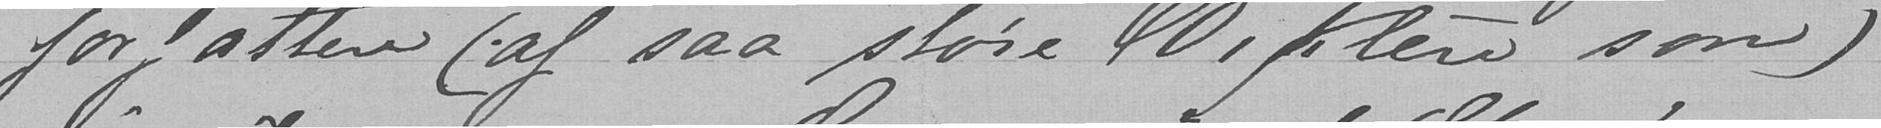forfattere (af saa store Diktere, som)
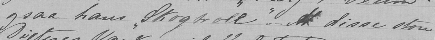og saa hans "Skogtoll". At disse store
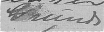Grunde
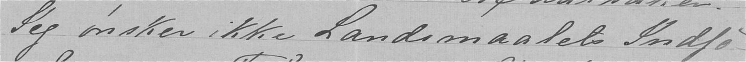Jeg ønsker ikke Landsmaalets Indfø-
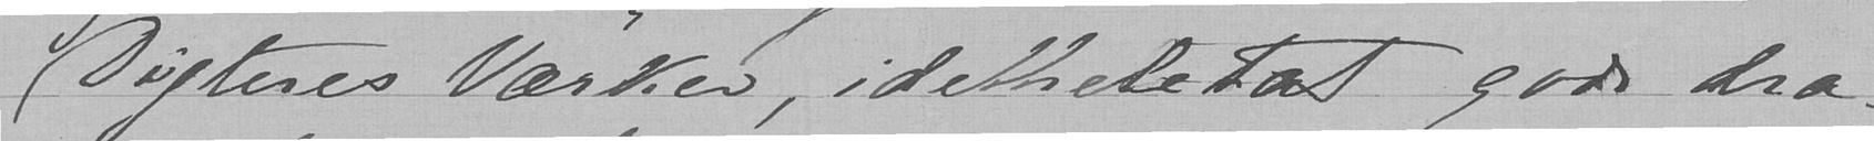Digteres Værker, idetheletat gode dra-
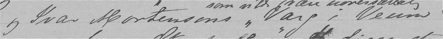og Ivar Mortensons "Varg i Veum"
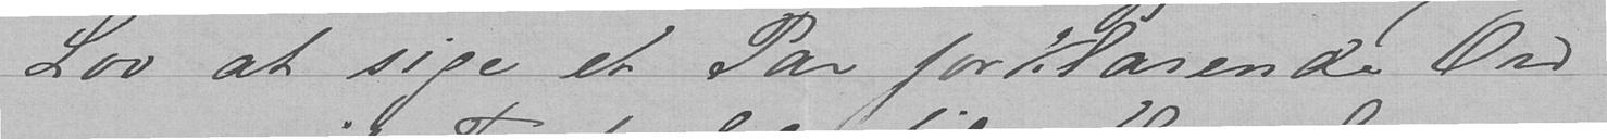Lov at sige et Par forklarende Ord
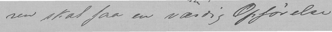ren skal faa en værdig Opførelse
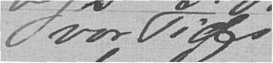vor Tids
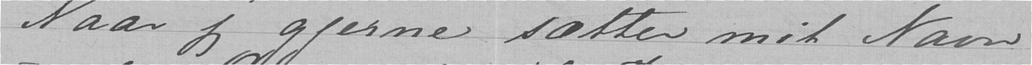Naar jeg gjerne sætter mit Navn
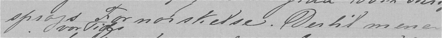sprogs Fornorskelse. Dertil mener
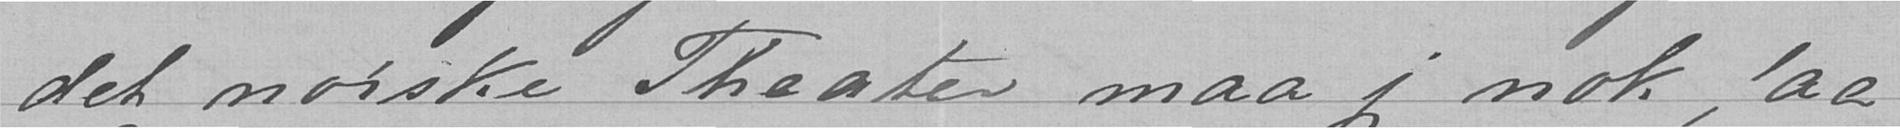det norske Theater maa jeg nok faa
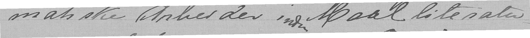matiske Arbeider inden Maal literatu-
